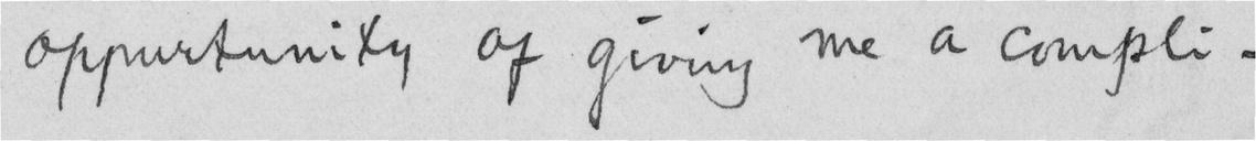oppurtunity of giving me a compli-
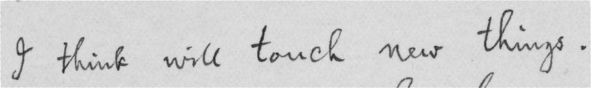I think will touch new things.
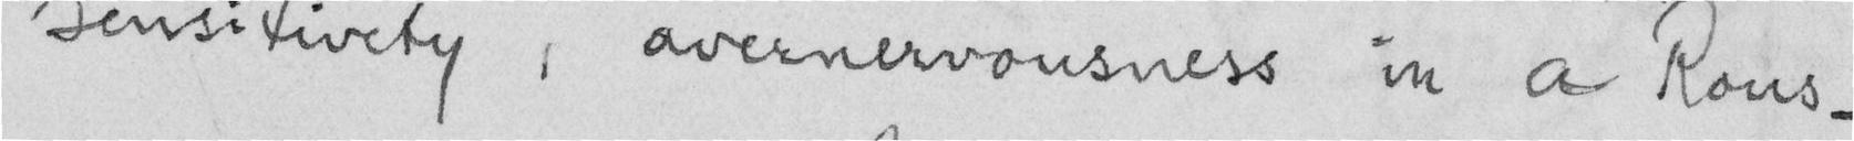sensitivety, overnervousness in a Rous-
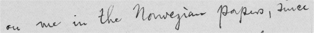on me in the Norwegian papers, since
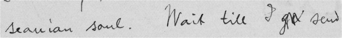seauian soul. Wait till I get send
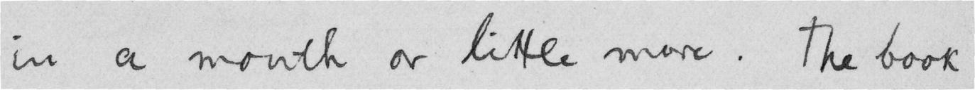in a month or little more. The book
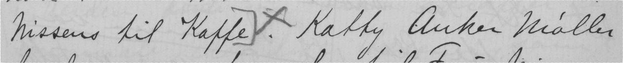Nissens til Kaffe. Katty Anker Møller
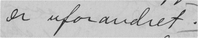er uforandret.
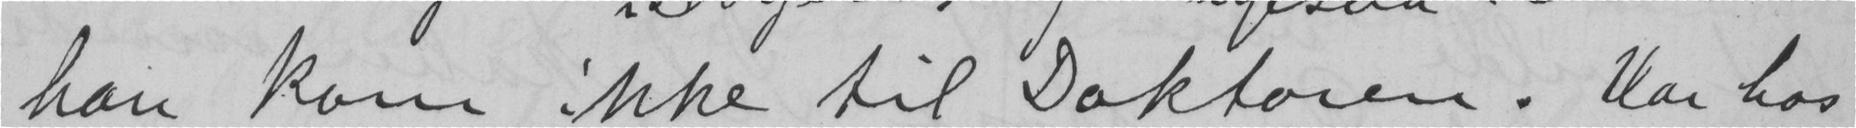han kom ikke til Doktoren. Var hos
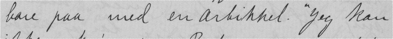bare paa med en artikkel. "Jeg kan
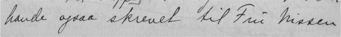havde ogsaa skrevet til Fru Nissen
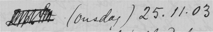(onsdag) 25.11.03
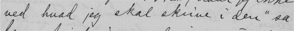ved hvad jeg skal skrive i den" sa
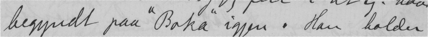begyndt paa "Boka" igjen. Han holder
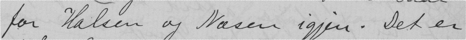for Halsen og Næsen igjen. Det er
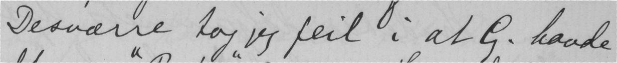Desværre tog jeg feil i at G. havde
